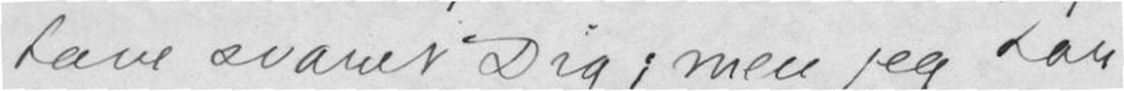have svaret Dig; men jeg har
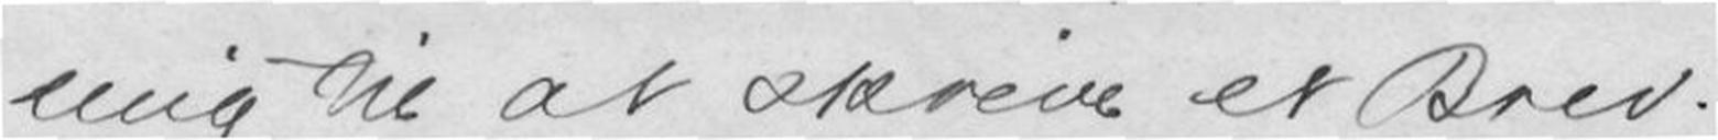mig til at skrive et Brev.
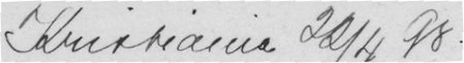Kristiania 22/4 98
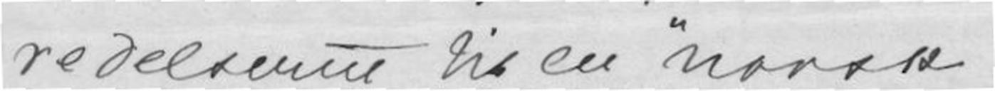redelserne til en "norsk
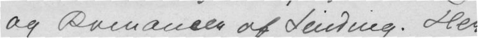og Romancen af Leidzing. Her
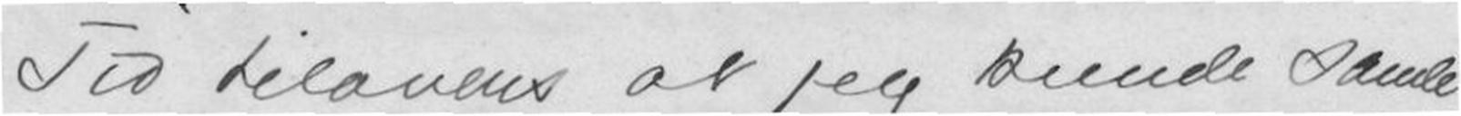Til tilovers at jeg kunde Sande
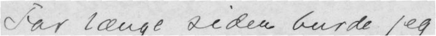For længe siden burde jeg
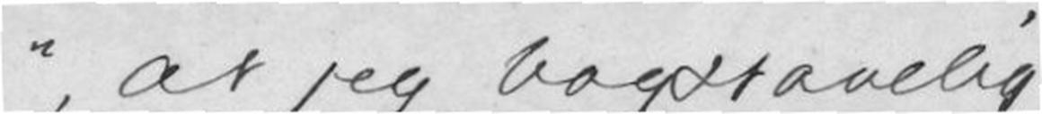", at jeg bogstavelig
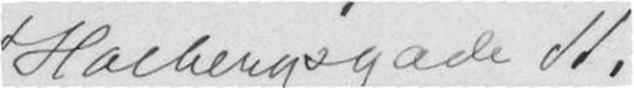Holbergsgade 11.
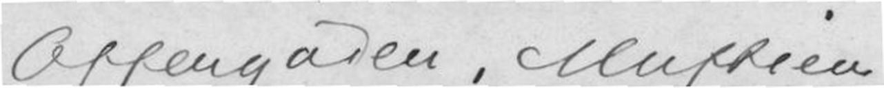Offergaven, Matthieu
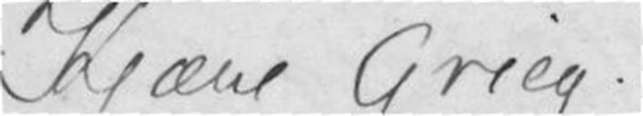Kjære Grieg
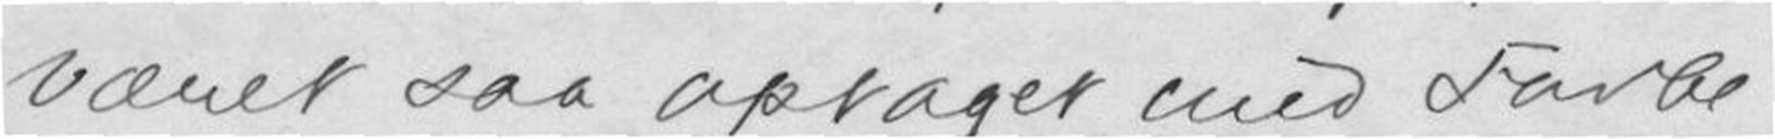været saa optaget med Forbe
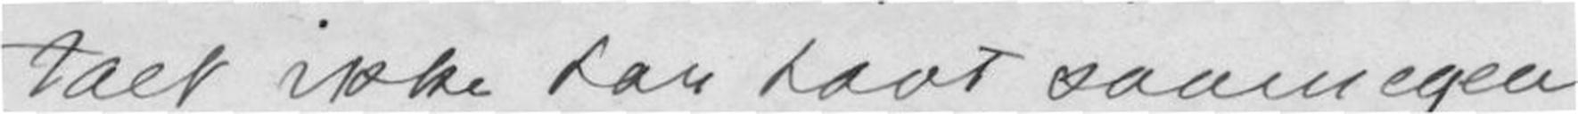talt ikke kan havt saamegen
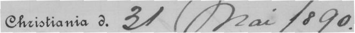Christiania d. 31 Mai 1890.
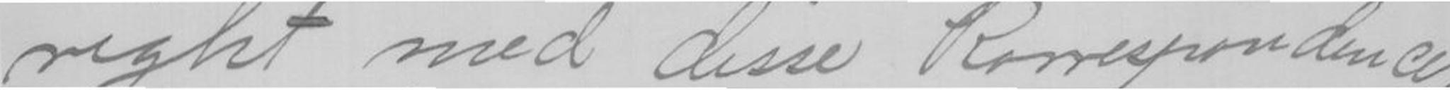right med disse korrespondence
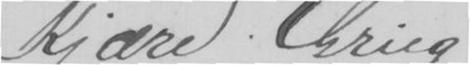Kjære Grieg
